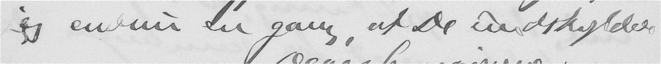jeg endnu en gang, at De undskylder
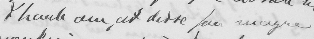I haab om, at disse faa magre
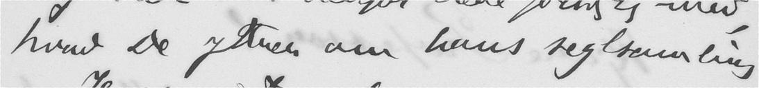hvad De yttrer om hans seglsamling.
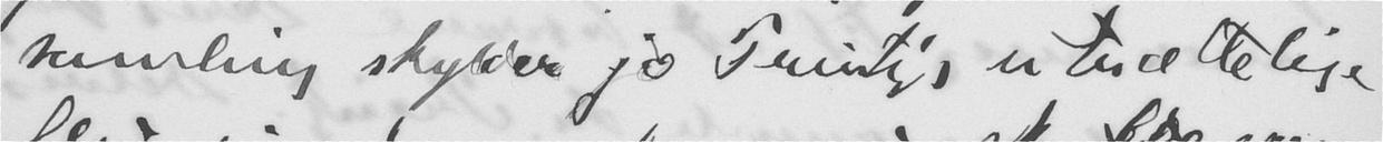samling skylder jo Printz's utrættelige
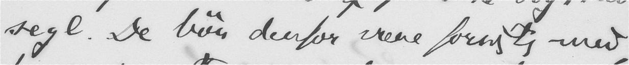segl. De bør derfor være forsigtig med
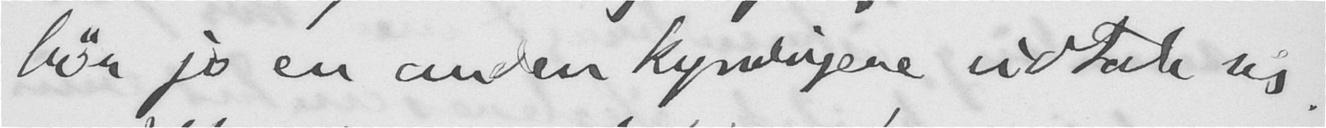bør jo en anden kyndigere udtale sig.
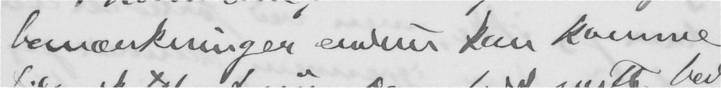bemærkninger endnu kan komme
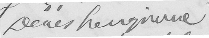Deres hengivne
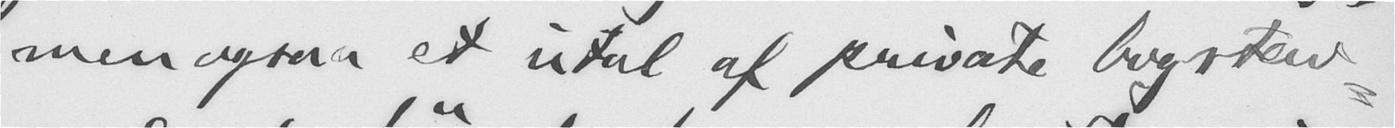men ogsaa et utal af private bogstav-
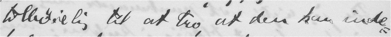tilbøielig til at tro, at den kan inde-
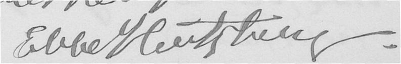Ebbe Hertzberg.
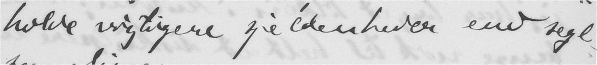holde vigtigere sjeldenheder end segl-
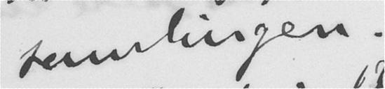samlingen.
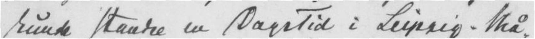kunde standse en Dagstid i Leipzig Må-
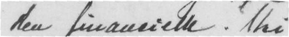den financielle. Thi
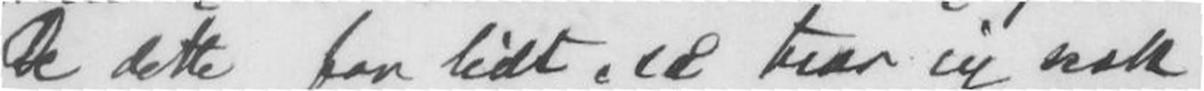De dette for lidet, så tror jeg nok
